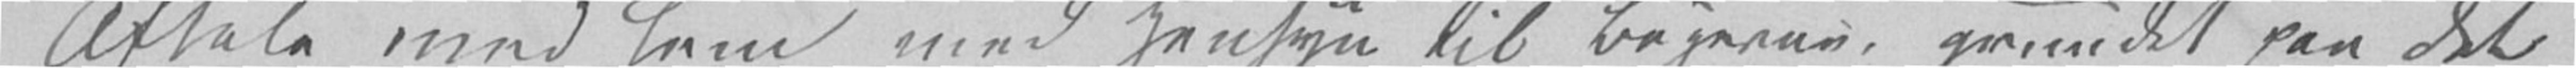Aftale med ham med Hensyn til Bøgerne, grundet paa det
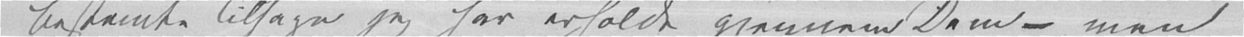bestemte Tilsagn jeg har erholdt gjennem Dem – men
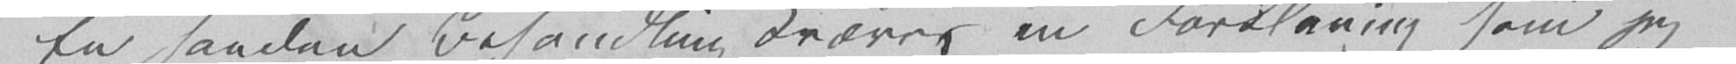En saadan Behandling kræver en Forklaring som jeg
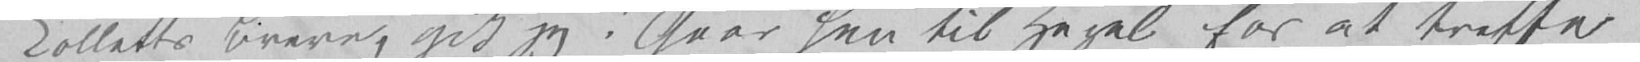Colletts Breve, gik jeg i Gaar hen til Hegel for at treffe
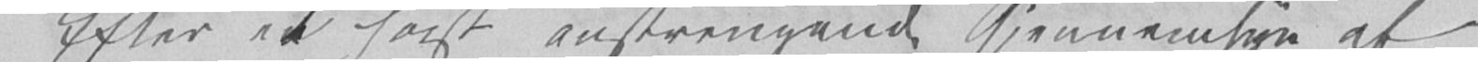Efter et høist anstrengende Gjennemsyn af
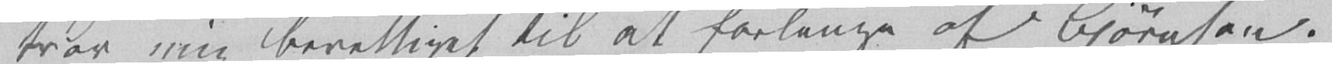tror mig berettiget til at forlange af Bjørnson.
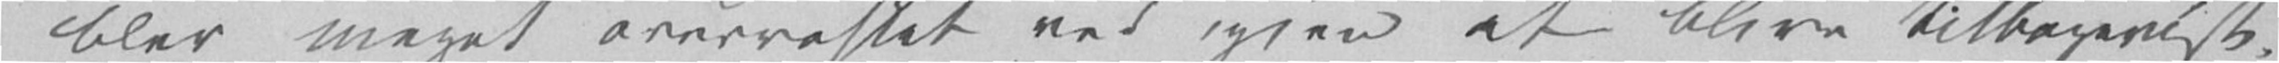blev meget overrasket ved igjen at blive tilbagevist.
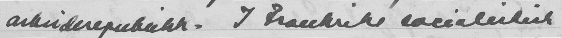arbeiderepublikk. I Frankrike socialistisk
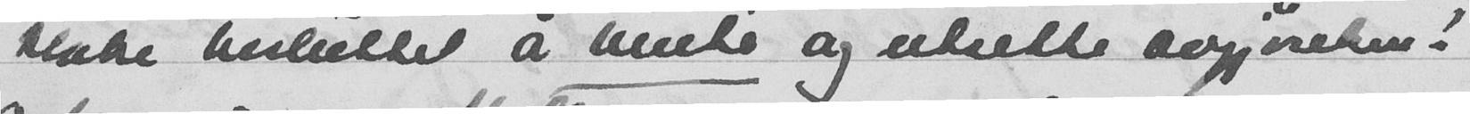kloke besluttet å vente og utsette avgjørelsen!
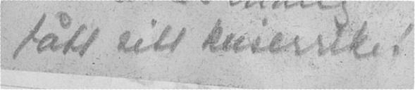fått sitt keiserrike!
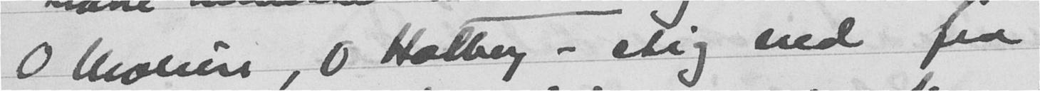O Moliere, O Holberg - stig ned fra
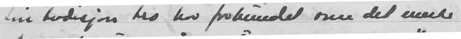Sin tradisjon tro har forbundet som det eneste
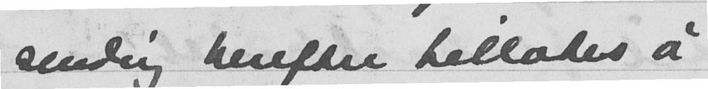vendig herefter tillates å
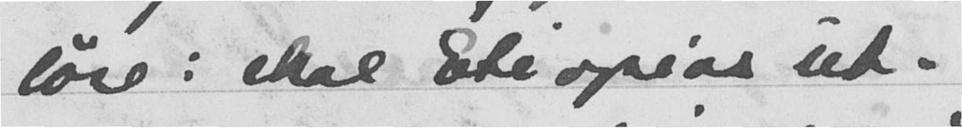løse; skal Etiopias ut-
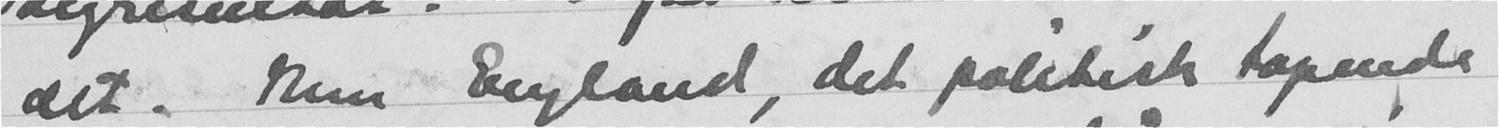det. Men England, det politisk tapende
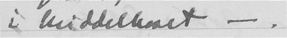i Middelhavet - .
